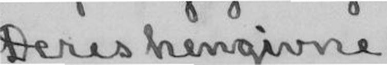Deres hengivne
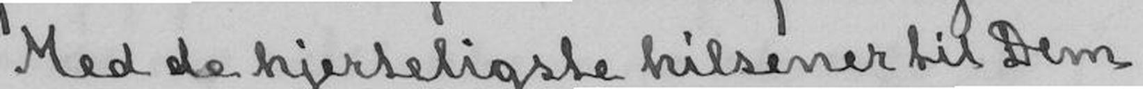Med de hjerteligste hilsener til Dem
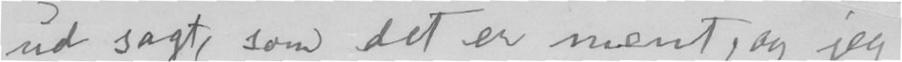ud sagt, som det er ment, og jeg
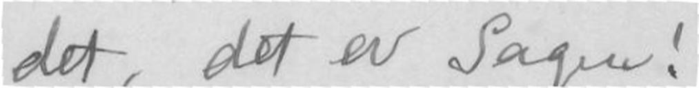det, det er Sagen!
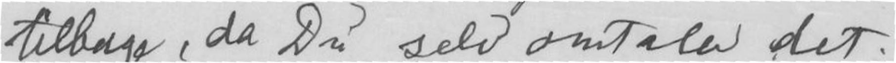tilbage, da Du selv omtaler det.
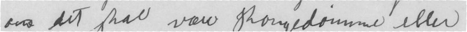om det skal være Kongedømme eller
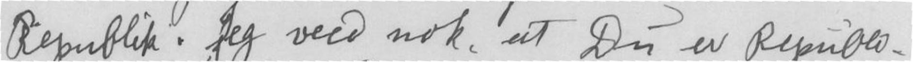Republik. Jeg veed nok at Du er Republi-
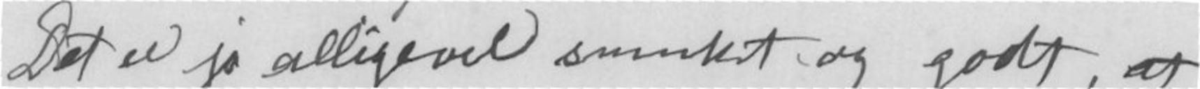Det er jo alligevel smukt og godt, at
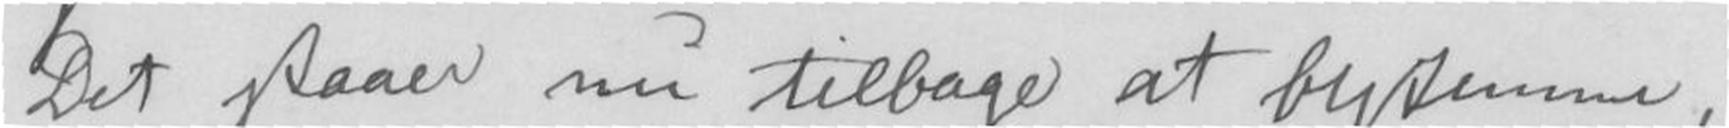Det staaer nu tilbage at bestemme,
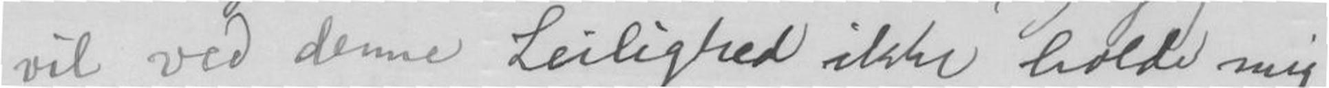vil ved denne Leilighed ikke holde mig
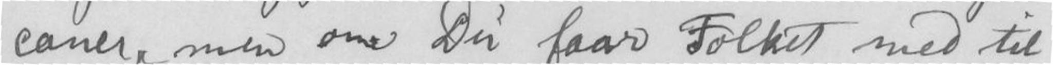caner, men om Du faar Folket med til
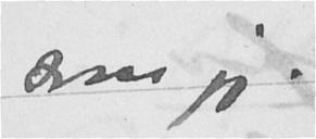som jeg.
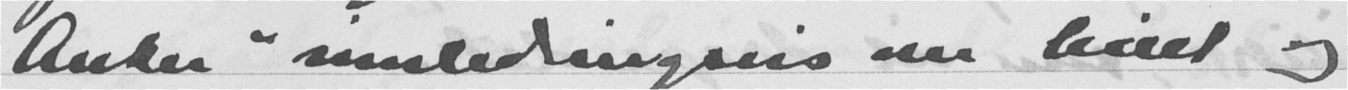Anker" innledningsvis om livet og
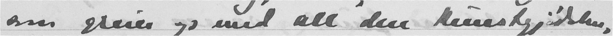som greier op med all den kunstgjødselen,
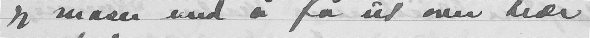jeg maser med å få ut over trær
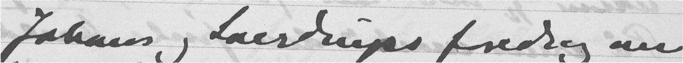Johans og Sverdrups foredrag om
